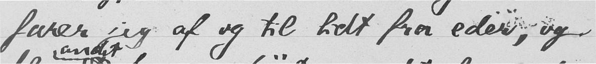farer jeg af og til lidt fra eder, og
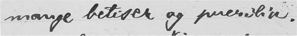mange betiser og puerilia.
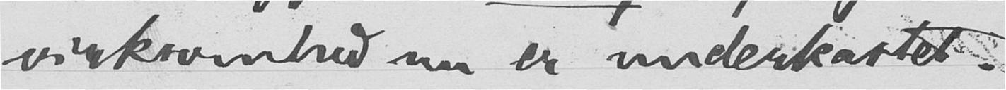virksomhed nu er underkastet.
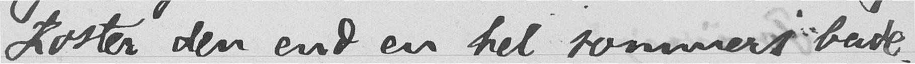Koster den end en hel sommers bade-
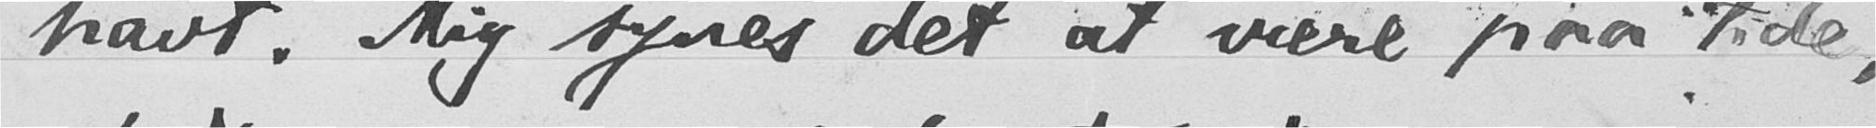havt. Mig synes det at være paa tide,
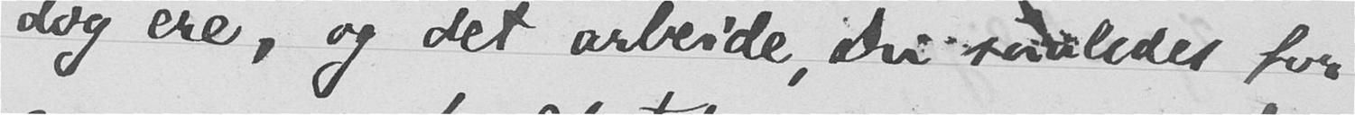dog ere, og det arbeide, Du saaledes for
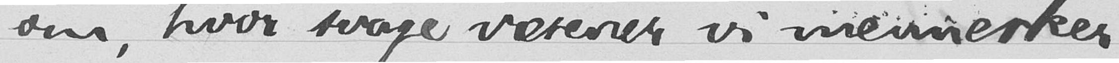om, hvor svage væsener vi mennesker
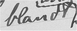blandt
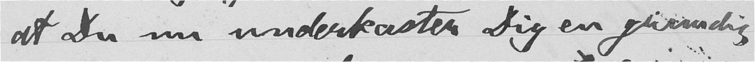at Du nu underkaster Dig en grundig
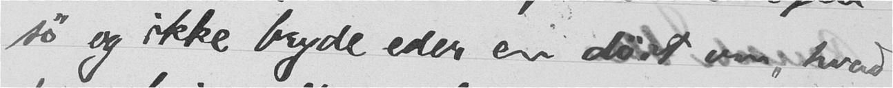sø og ikke bryde eder en døit om, hvad
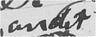andet
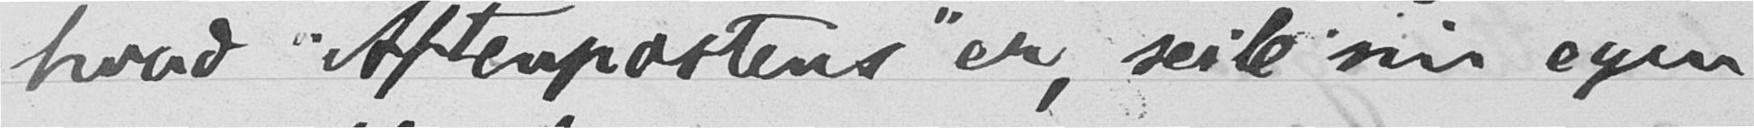hvad "Aftenpostens" er, seile sin egen
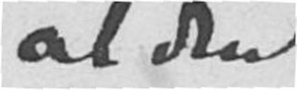al den
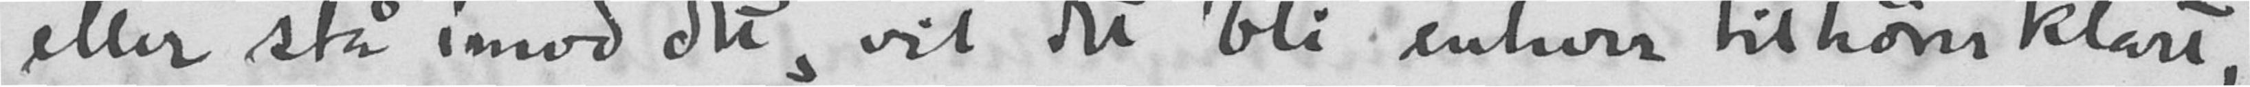eller stå imod det, vil det bli enhver tilhører klart,
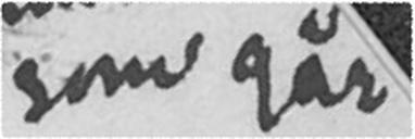som går
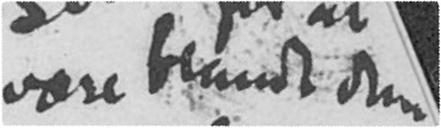være blandt dem
